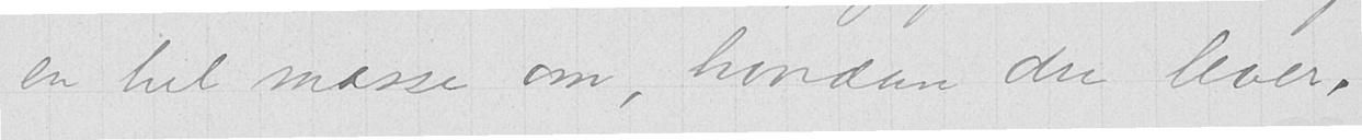en hel masse om, hvordan du lever,
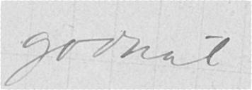godnat.
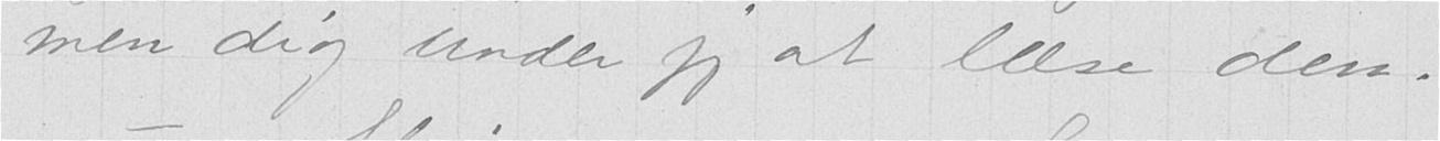men dig under jeg at læse dem.
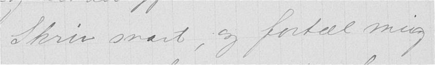— Skriv snart, og fortæl mig
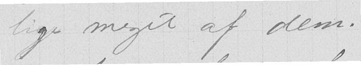lige meget af dem.
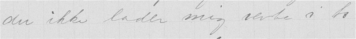du ikke lader mig vente i to
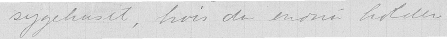sygehuset, hvis du endnu holder
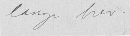brev.  S.
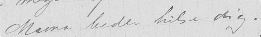Mama beder hilse dig.
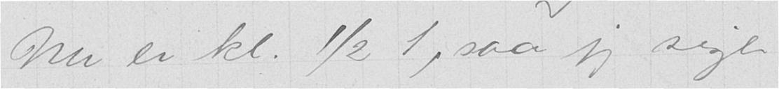Nu er kl. ½ 1, saa jeg siger
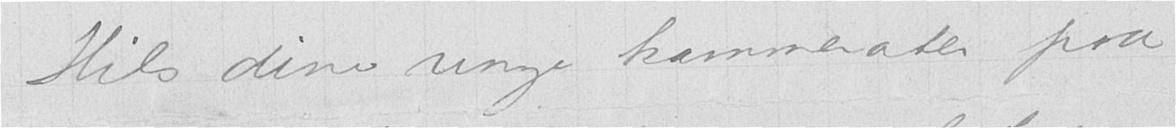Hils dine unge kammerater paa
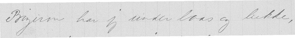Bøgerne har jeg under laas og lukke,
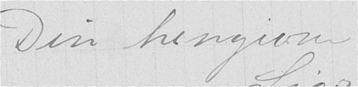Din hengivne
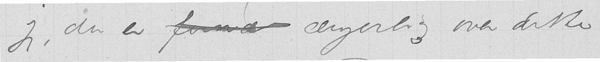jeg du er fan ærgerlig over dette lange
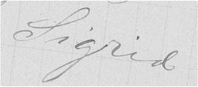Sigrid.
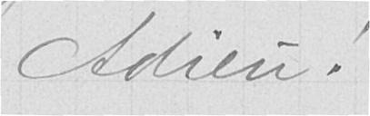Adieu!
